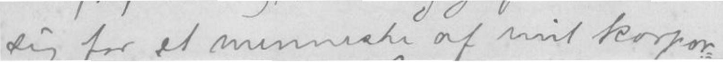sig for et menneske af mit korpor-
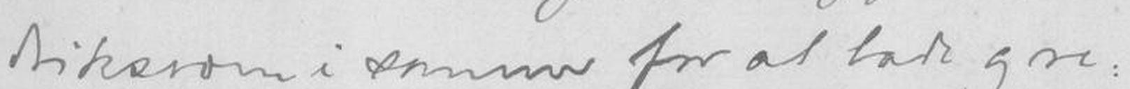driksrom i sommer for at bade og re-
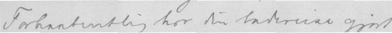Forhaabentlig har din badereise gjort
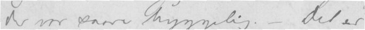der var saare hyggelig.- det er
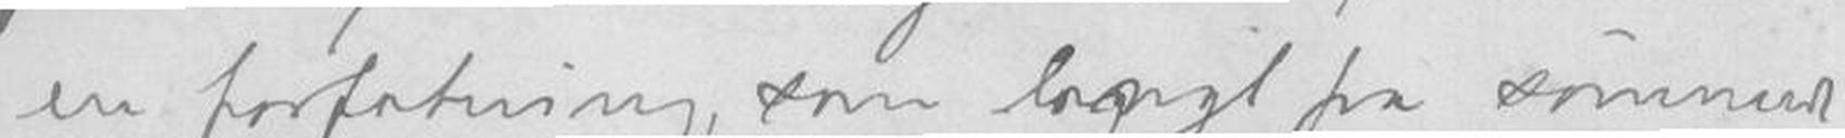en forfatning, som langt fra sømmede
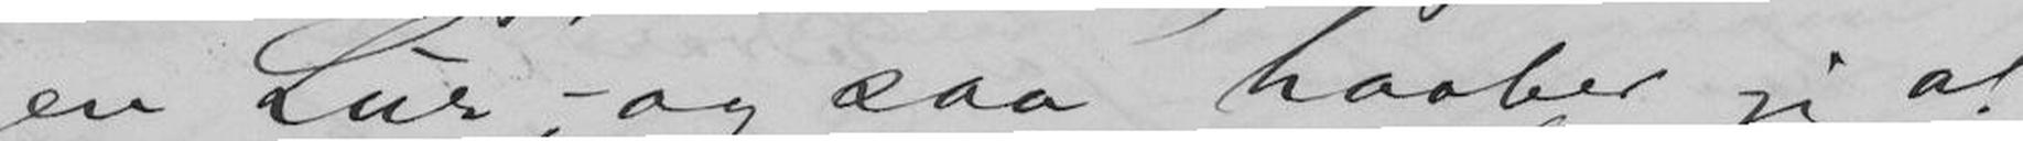en Lur, - og saa haaber jeg at
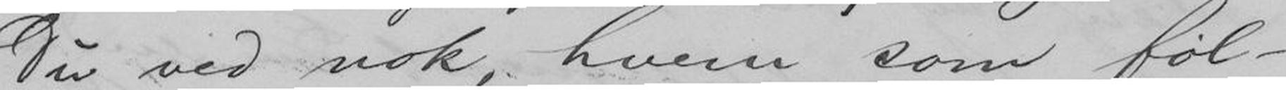Du ved nok, hvem som føl-
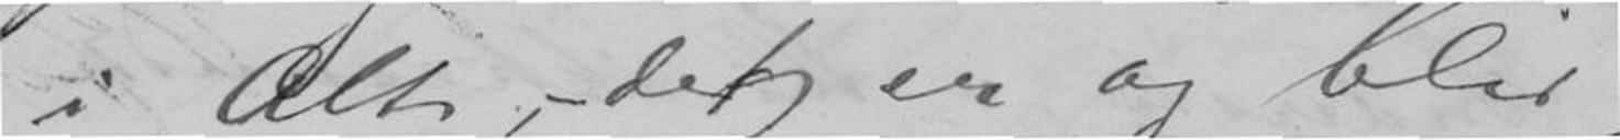i Alt, det er og blir
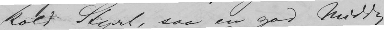kold Styrt, saa en god Middag
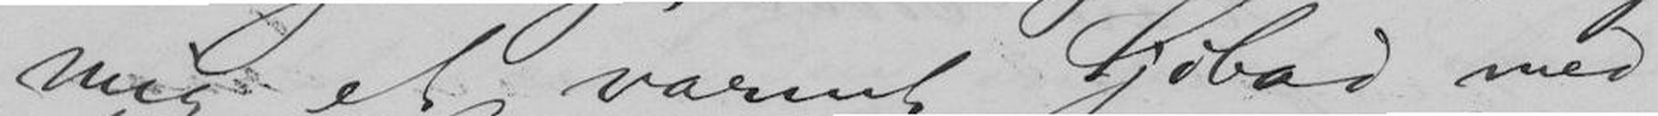mig et varmt Sjøbad med
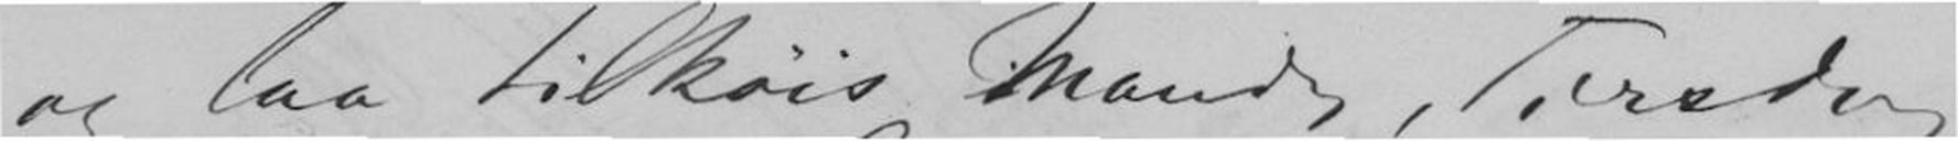og laa tilkøis Mandag, Tirsdag
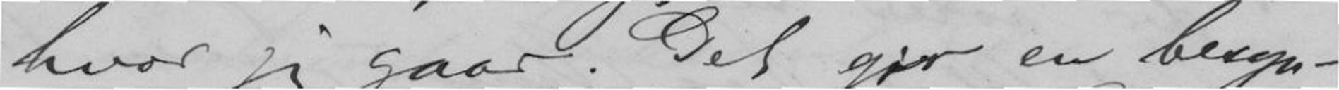hvor jeg gaar. Det gir en besyn-
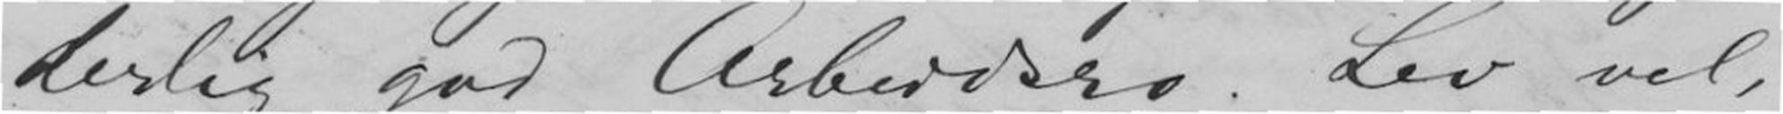derlig god Ardeidsro. Lev vel,
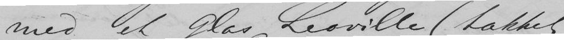med et glas Leoville (takket
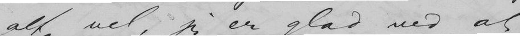alt vel, jeg er glad ved at
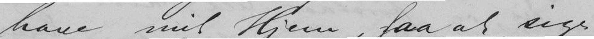have mit Hjem, saa at sige
